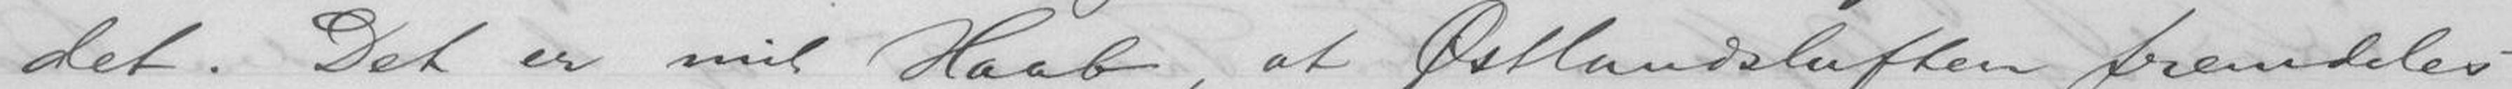det. Det er mit Haab, at Østlandsluften fremdeles
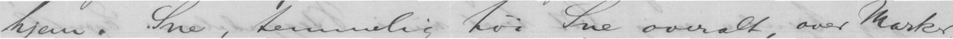hjem. Sne, temmelig høi Sne overalt, over Marker,
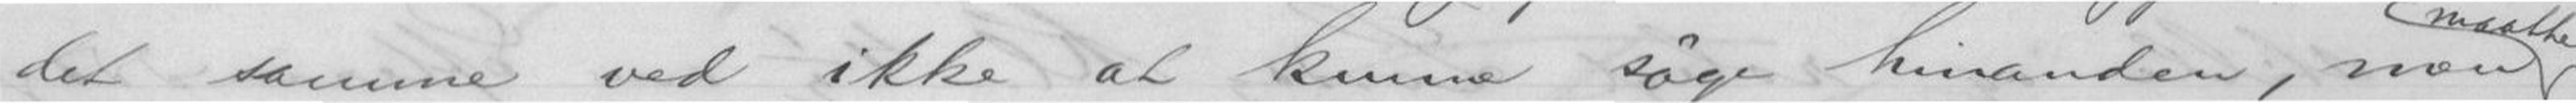det samme ved ikke at kunne søge hinanden, men
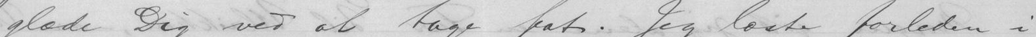glæde Dig ved at tage fat. Jeg læste forleden i
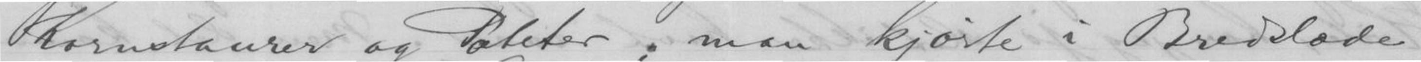Kornstaurer og Poteter; man kjørte i Bredslæde,
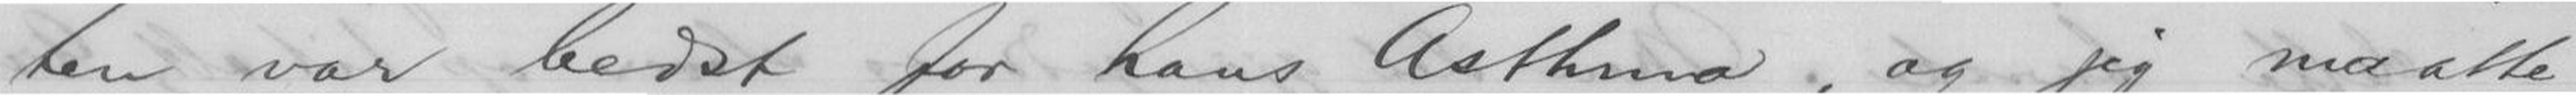ten var bedst for hans Asthma, og jeg maatte
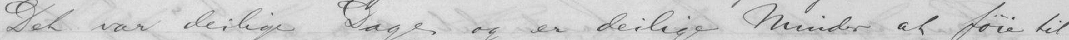Det var deilige Dage og er deilige Minder at føie til
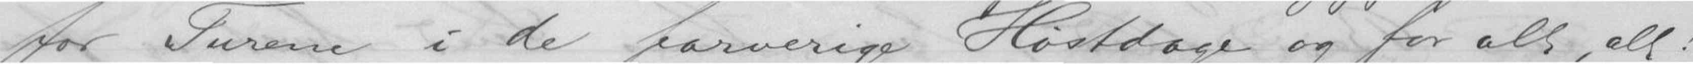for Turene i de farverige Høstdage og for alt, alt!
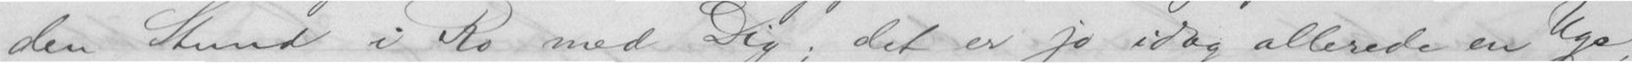den Stund i Ro med Dig; det er jo idag allerede en Uge
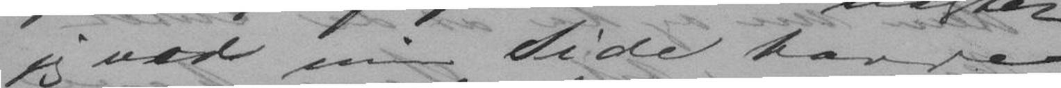jeg ved min Side havde
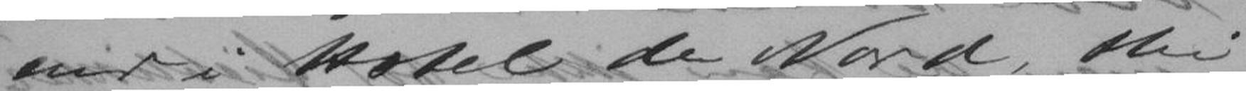end i Hotel de Nord, thi
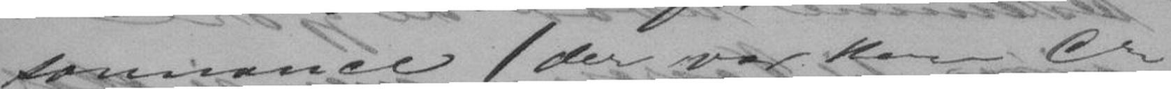sonnance (der var kun Cr
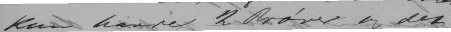kun havde 2 Prøver og det
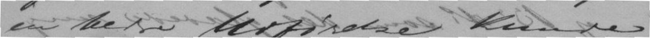en bedre Udførelse kunde
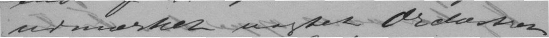udmærket uagtet Orchestret
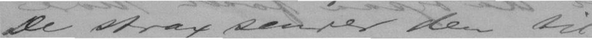De strax sender den til
